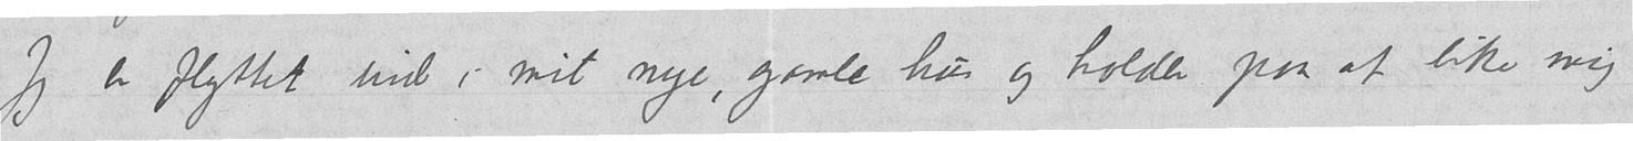Jeg er flyttet ind i mit nye, gamle hus og holder paa at like mig
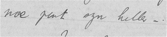noe pent syn heller –.
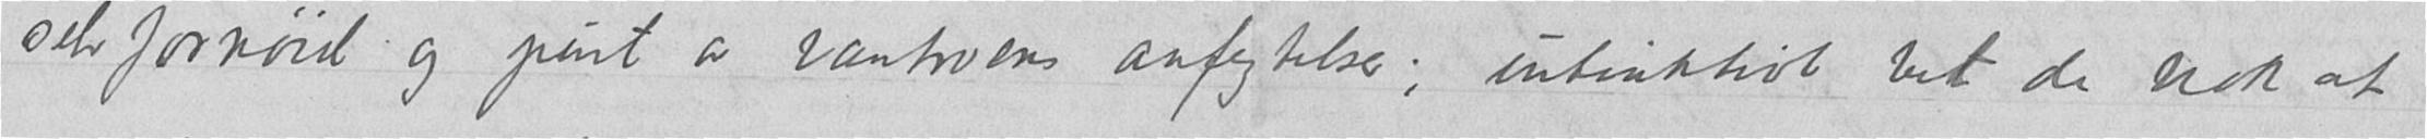selvfornøiet og pint av vantroens anfegtelser; instinktivt vet de nok at
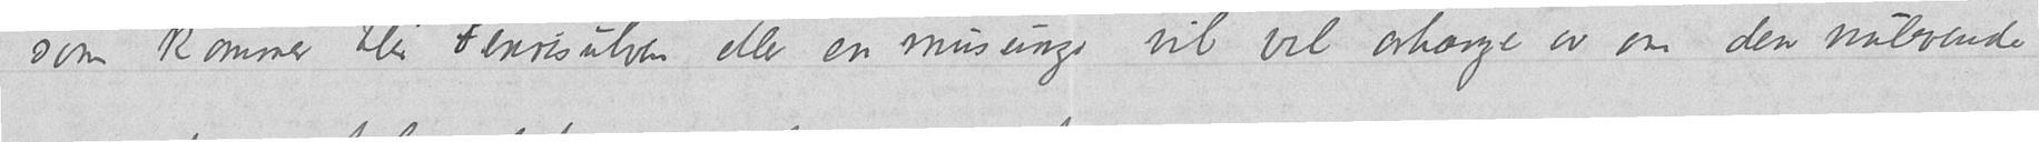som kommer blir Fenrisulven eller en musunge vil vel avhænge av om den nulevende
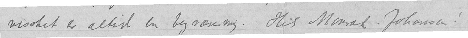visshet er altid en begrænsmig. Hils Monrad-Johansen!
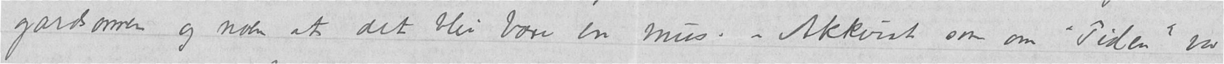gardsormen og noen at det blir bare en mus. – Akkurat som om "Tiden" var
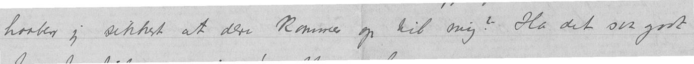haaber jeg sikkert at dere kommer op til mig? Ha det saa godt
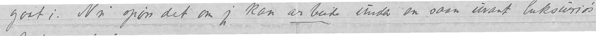gaat i. Nu spørs det om jeg kan arbeide under en saan uvant luksuriøs
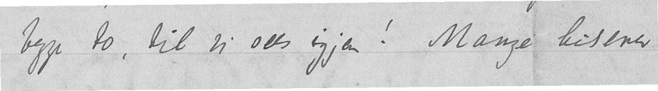begge to, til vi sees igjen! Mange hilsener
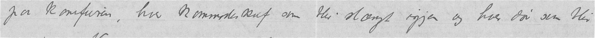paa komfuren, hver kommodeskuf som blir slængt igjen og hver dør som blir
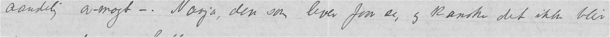aandelig avmagt –. Naaja, den som lever faar se, og kanske det ikke blir
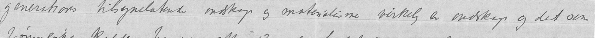generations tilsynelatende ondskap og materialisme virkelig er ondskap og det som
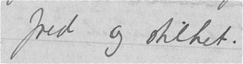fred og stilhet.
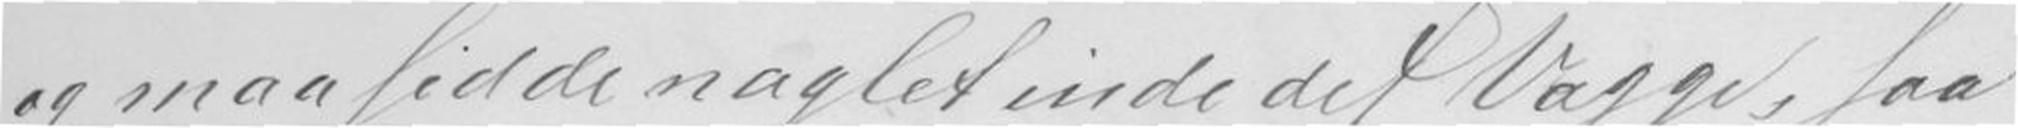og maa Sidde naglet inde de faa
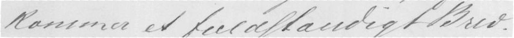kommer et fuldstændigt Brev.
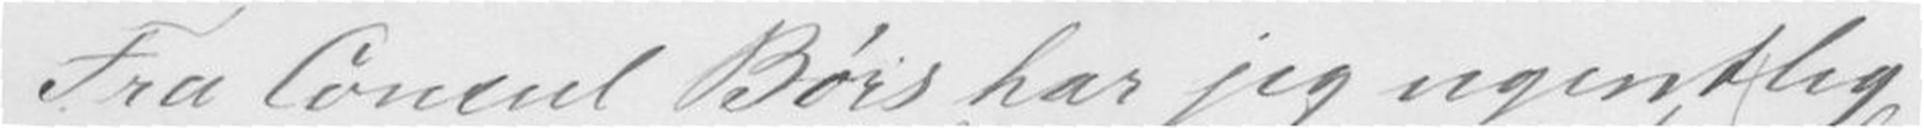Fra Concul Børs har jeg egentlig
